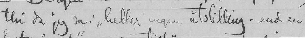thi da jeg sa: "heller ingen utstilling - end en
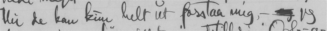thi de kan kun helt ut forstaa mig, -  jeg
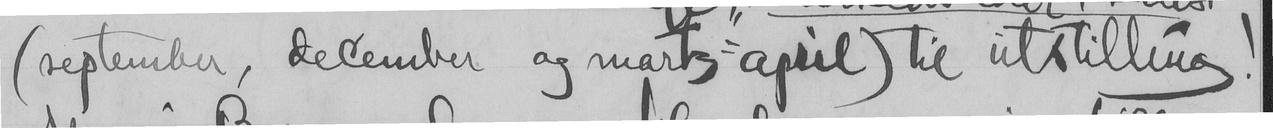september, december og martz - april) til utstilling!
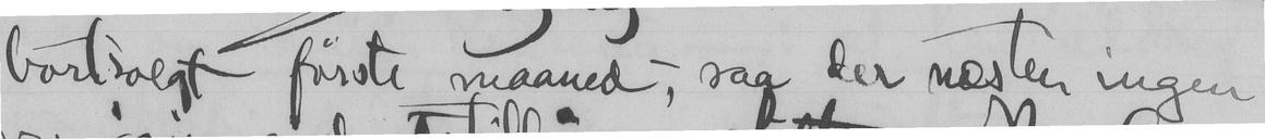bortsolgt første maaned , saa der næsten ingen
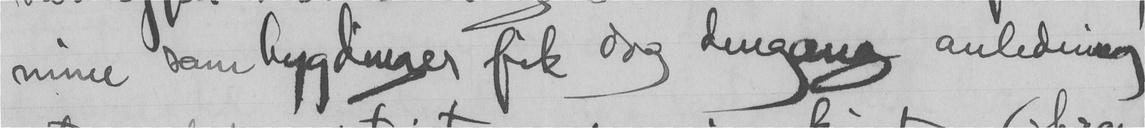mine sambygdinger fik dog dengang anledning
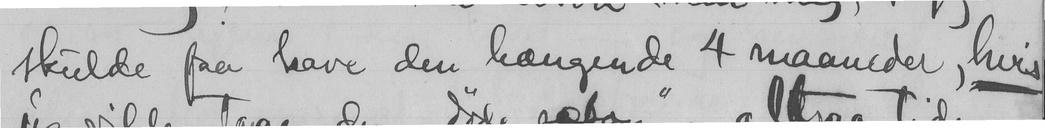skulde faa have den hængende 4 maaneder, hvis
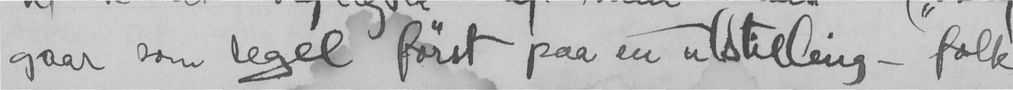gaar som regel først paa en utstilling - folk
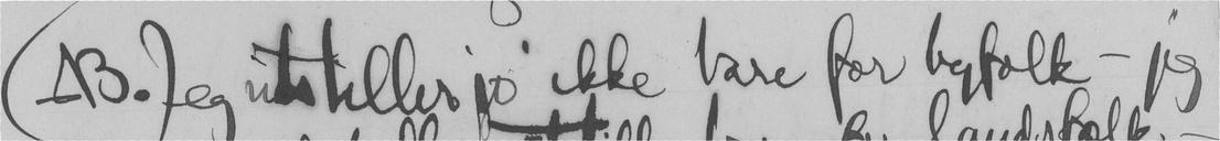(NB. Jeg utstiller jo ikke bare for byfolk - jeg
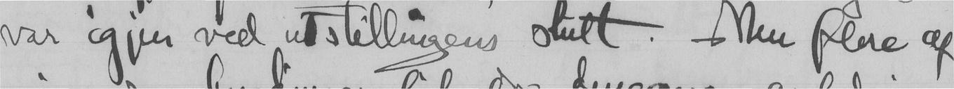var igjen ved udstillingens slutt. Men flere af
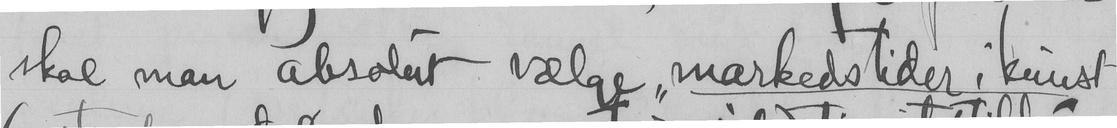skal man absolut vælge "markedstider i kunst" -
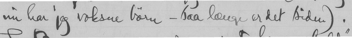nu har jeg voksne børn - (saa længe er det siden).
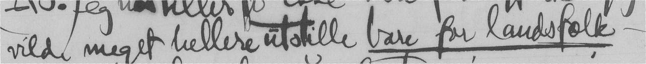vilde meget hellere utstille bare for landsfolk,
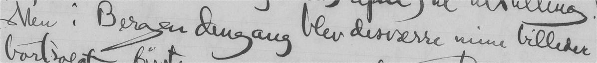Men i Bergen dengang blev desværre mine billeder
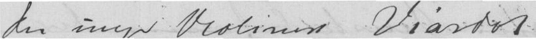Den unge Violinist Viardot
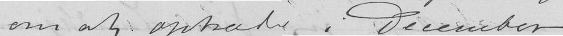om at optræde i December
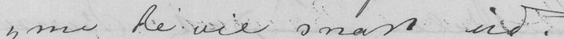me De vel snart ud. -
